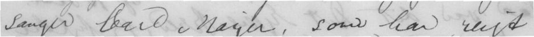sanger Carl Mayer, som har rejst
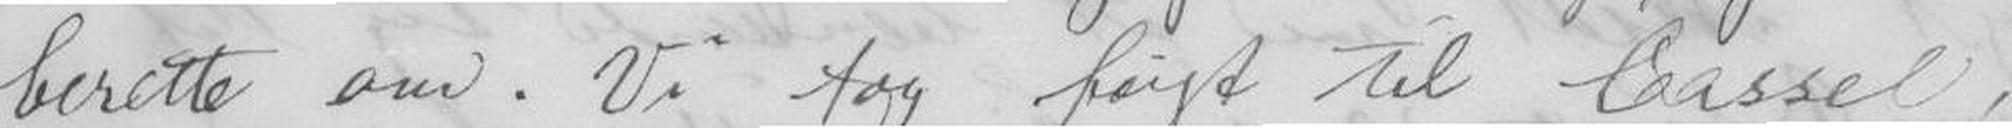berette om. Vi tog først til Cassel,
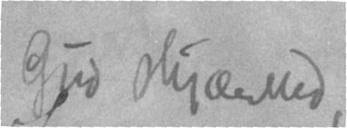Gud æ
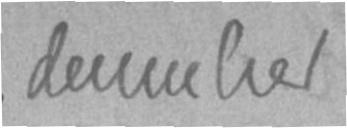denne her
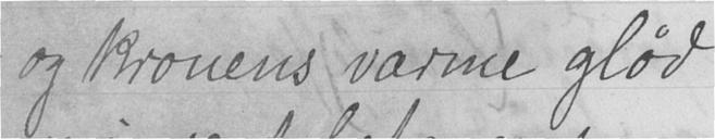og kronens varme glød
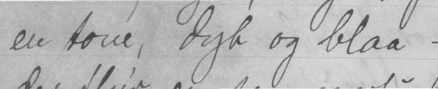en tone, dyb og blaa -
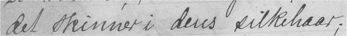det skinner i dens silkehaar;
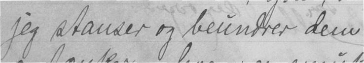jeg stanser og beundrer dem
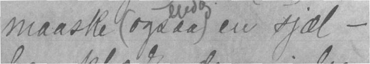maaske (ogsaa) endog en sjæl -
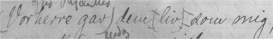(Vor herre gav) dem (liv) som mig,
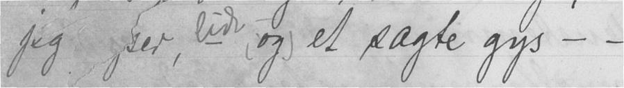jeg ser lidt, - (og) et sagte gys - -
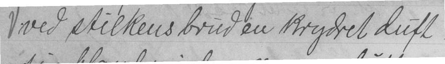V ved stilkens brud en krydret duft
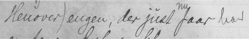(Henover) engen, der just nu faar har
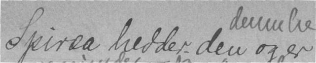Spiræa hedder den og er
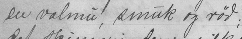en valmu, smuk og rød;
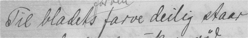Til bladets farve deilig staar
